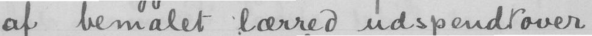af bemalet lærred udspendt over
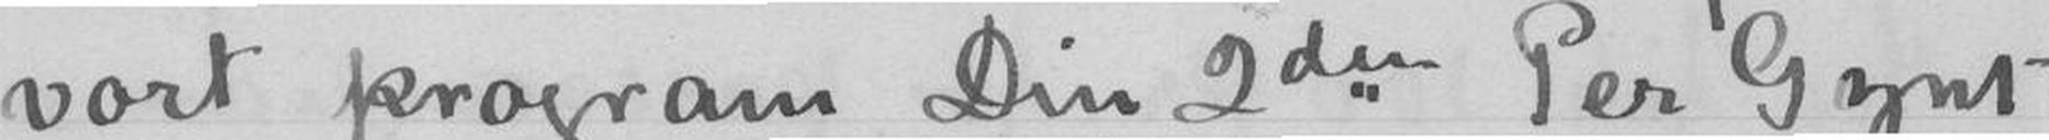vort program Din 2den Per Gynt
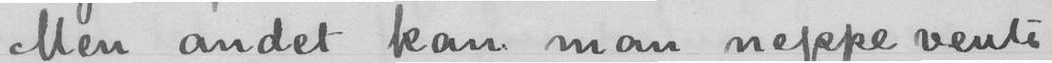Men andet kan man neppe vente
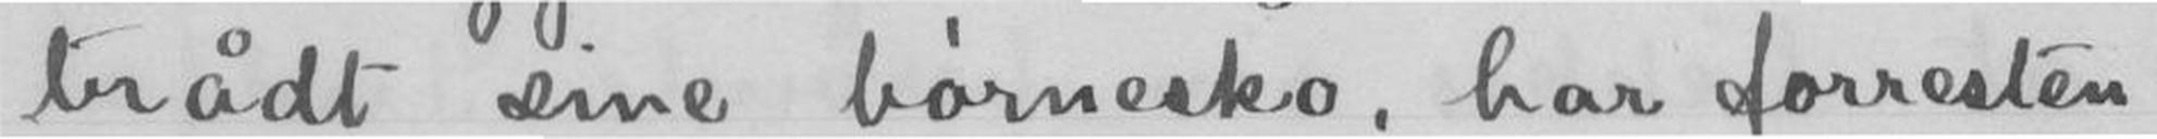trådt sine børnesko, har forresten
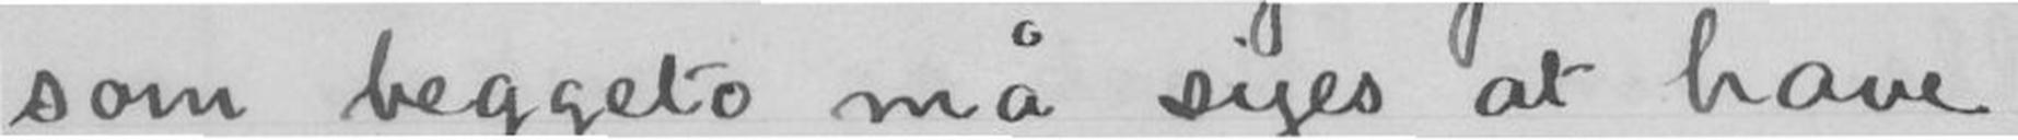som beggeto må siges at have
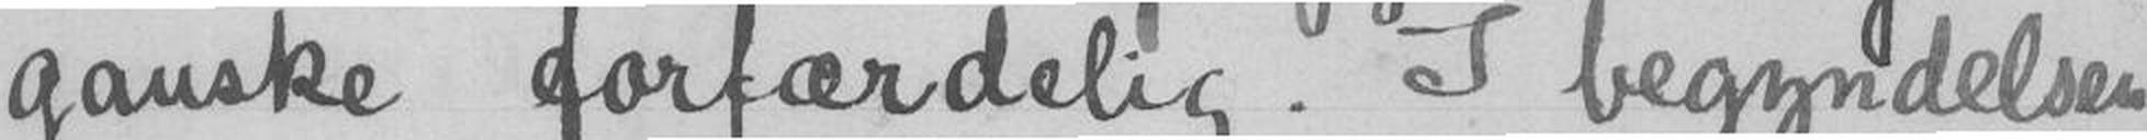ganske forfærdelig. I begyndelsen
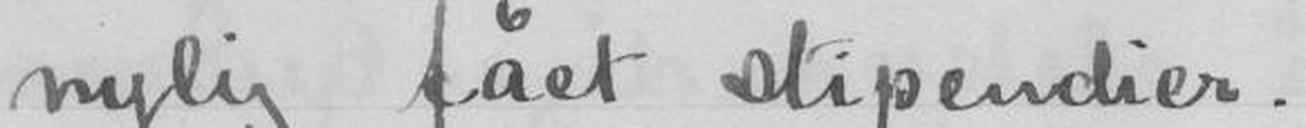nylig fået stipendier.
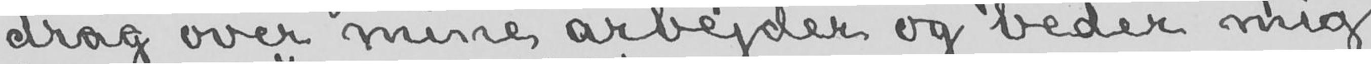drag over mine arbejder og beder mig
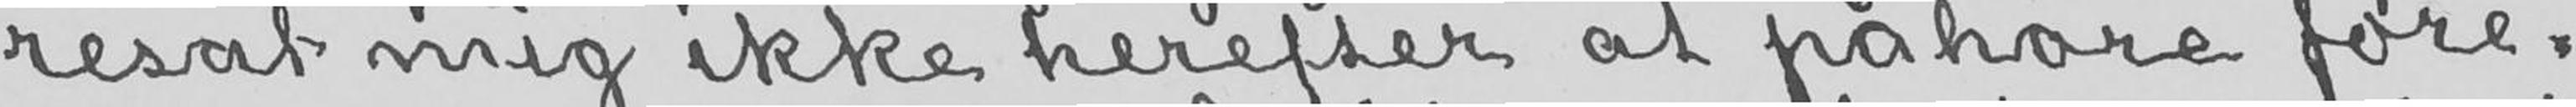resat mig ikke herefter at påhøre fore-
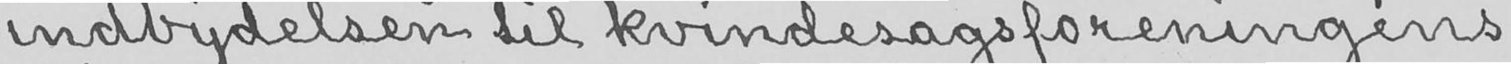indbydelsen til kvindesagsforeningens
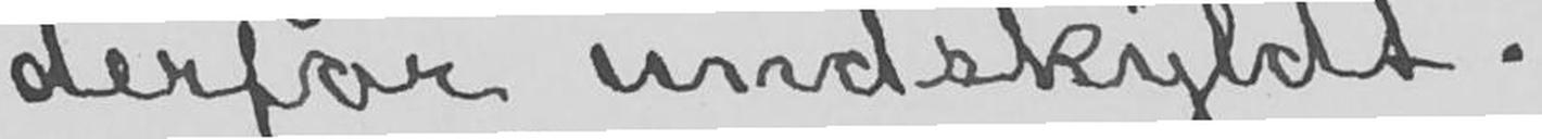derfor undskyldt.
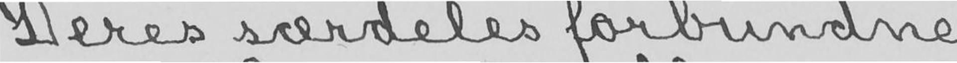Deres særdeles forbundne
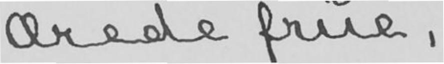Ærede frue,
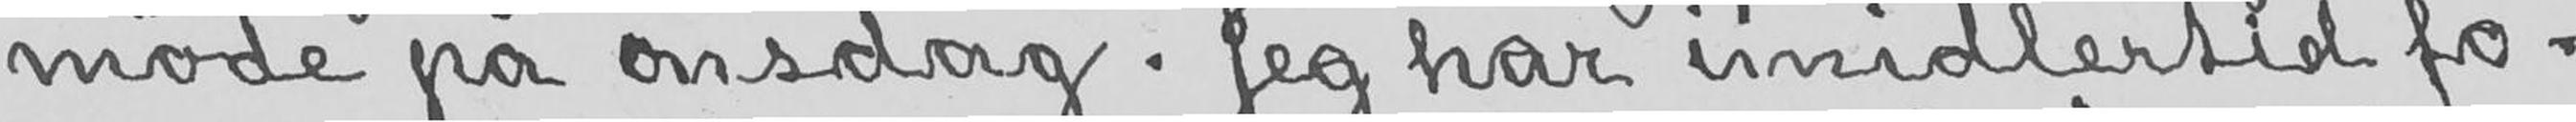møde på onsdag. Jeg har imidlertid fo-
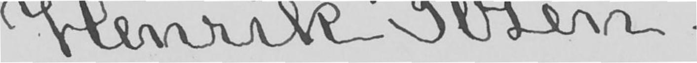Henrik Ibsen.
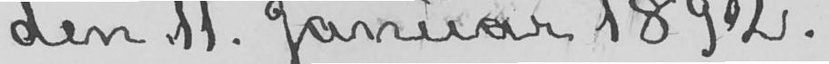den 11. Januar 1892.
